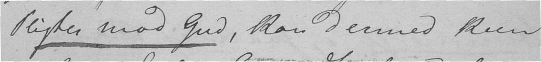Pligter mod Gud, kan dermed kun
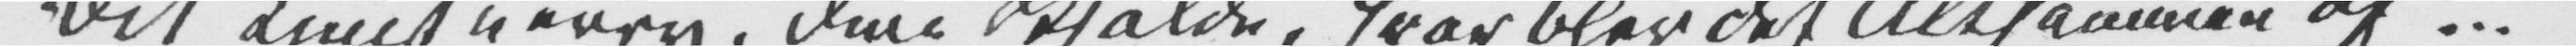Dit Kunstnerry, dine Skjalde, hvor blev det Altsammen af? ...
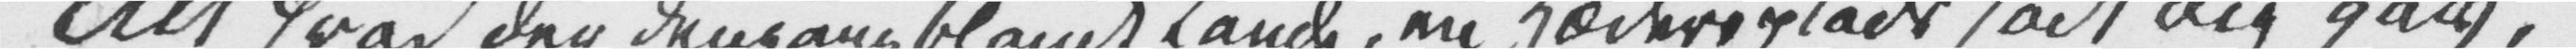Alt hvad der dengang blandt Lande, en Hædersplads høit dig gav,
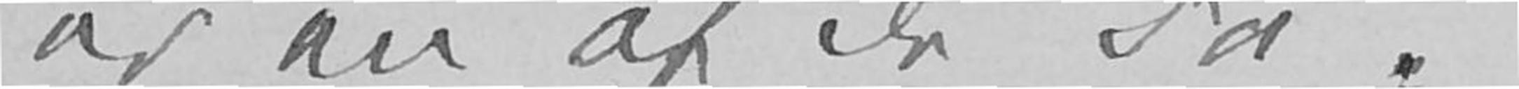er en af de Få.
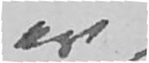er
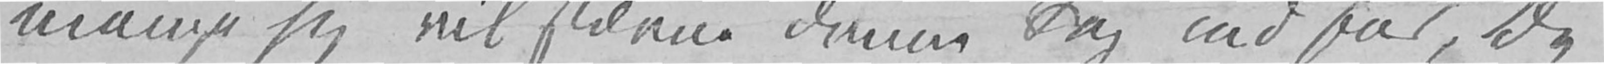mange jeg vil stævne denne Sag ind for, De
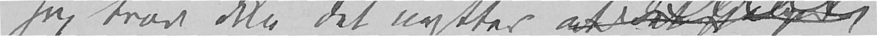Jeg tror ikke det nytter at det hjelper
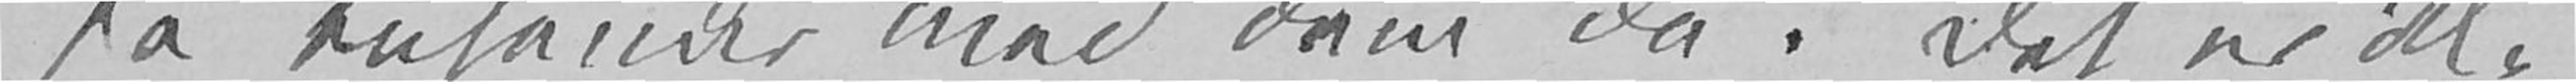få tusender med Dem da. Det er ikke
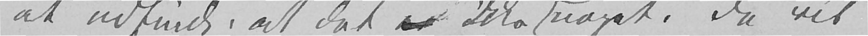at udfinde, «at det er ikke noget.» De vil
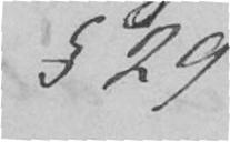§ 29
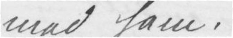med ham?
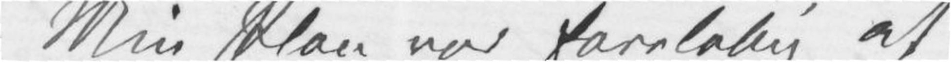Min Plan var foreløpig at
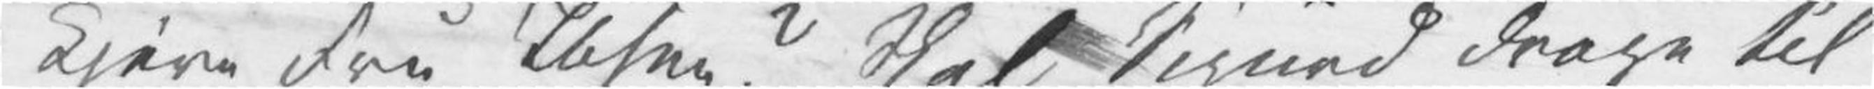kjere Fru Ibsen? Skal Sigurd drage til
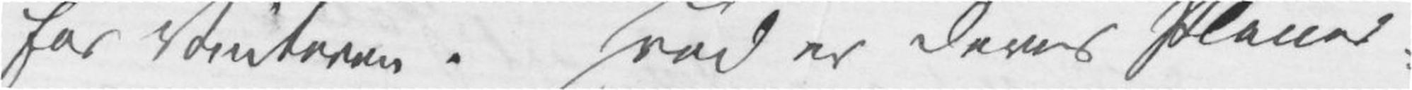for Vinteren. Hvad er Deres Planer
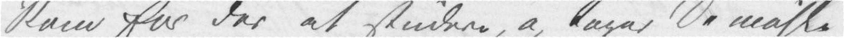Rom for der at studere, og tager De måske
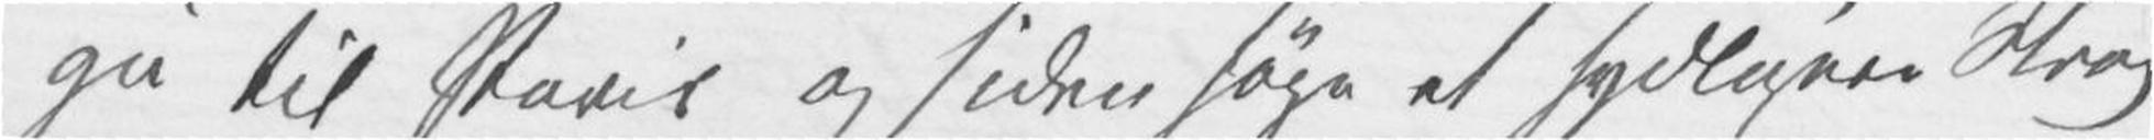gå til Paris og siden søge et sydligere Strøg
